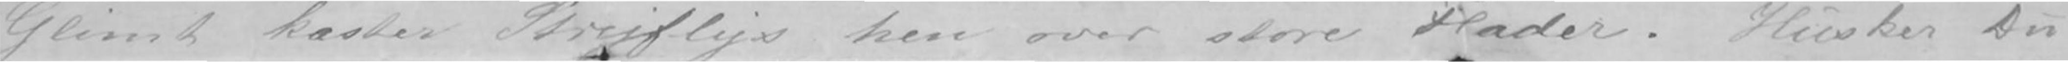Glimt kaster Strejflys hen over store Flader. Husker Du
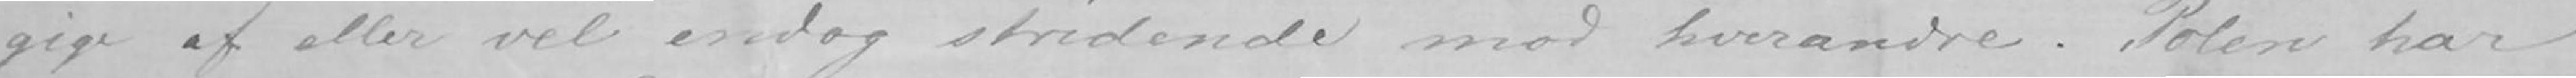gige af eller vel endog stridende mod hverandre. Polen har
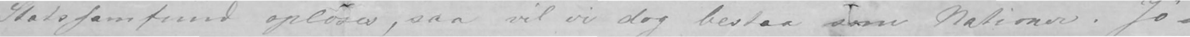Statssamfund opløses, saa vil vi dog bestaa som Nationer. Jø-
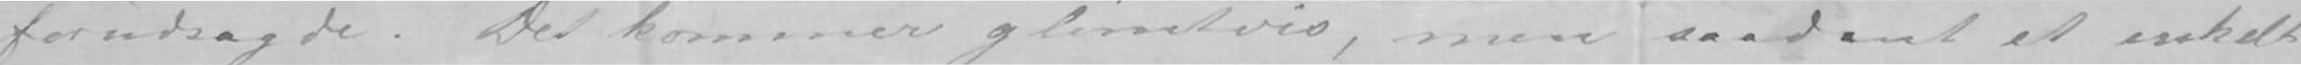forudsagde. Det kommer glimtvis, men saadant et enkelt
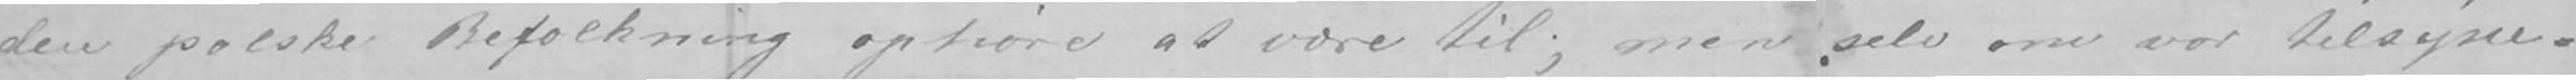den polske Befolkning ophøre at være til; men selv om vor tilsyne-
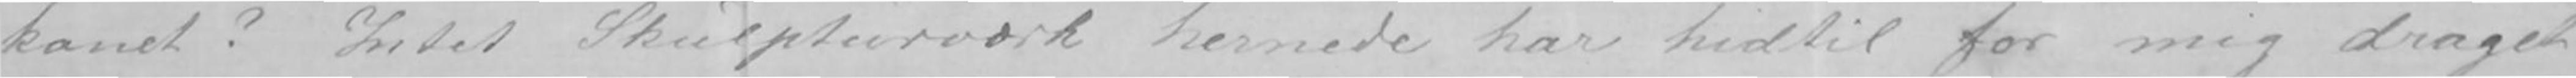kanet? Intet Skulpturværk hernede har hidtil for mig draget
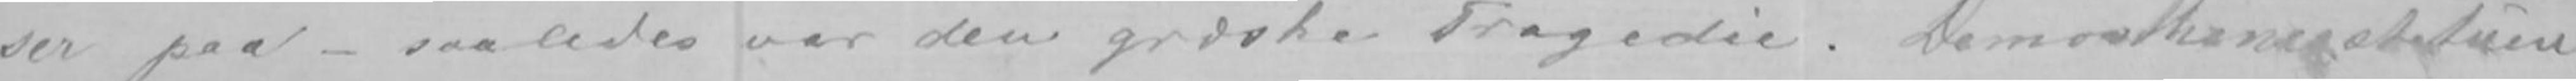ser paa- saaledes var den græske Tragedie. Demosthenesstatuen
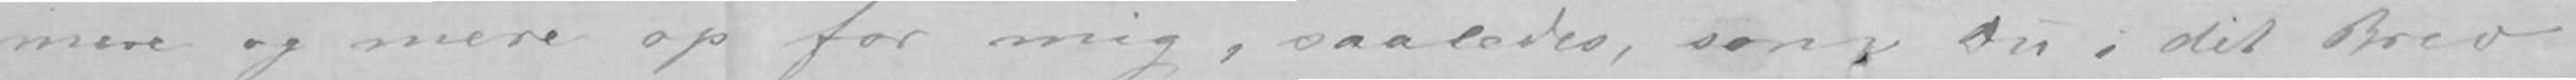mere og mere op for mig, saaledes, som Du i dit Brev
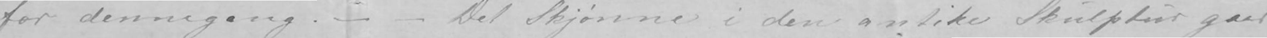for dennegang.-- Det Skjønne i den antike Skulptur gaar
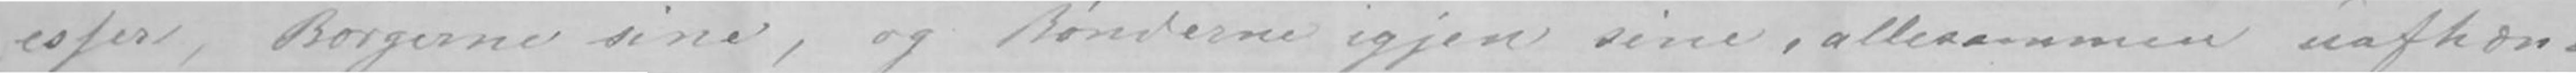esser, Borgerne sine, og Bønderne igjen sine, allesammen uafhæn-
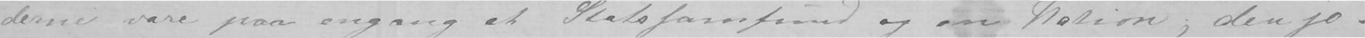derne vare paa engang et Statssamfund og en Nation; den jø-
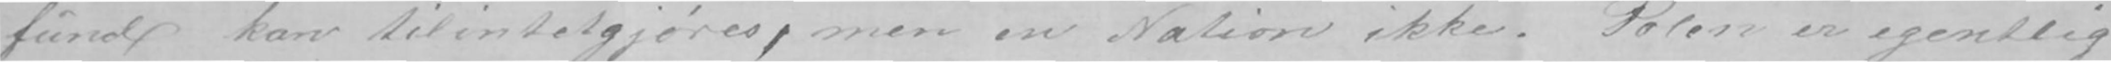fund kan tilintetgjøres, men en Nation ikke. Polen er egentlig
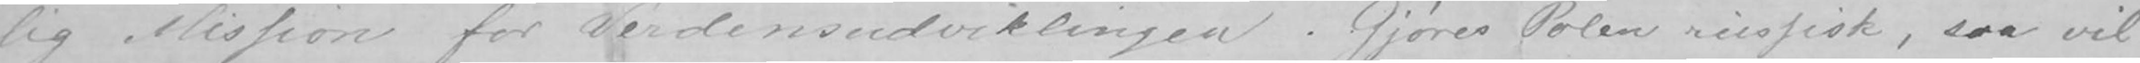lig Mission for Verdensudviklingen. Gjøres Polen russisk, saa vil
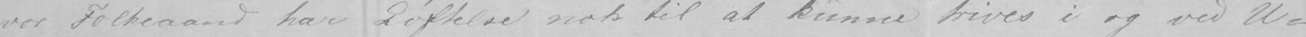vor Folkeaand har Løftelse nok til at kunne trives i og ved U-
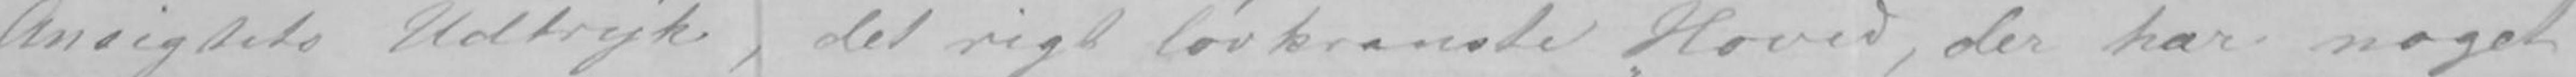Ansigtets Udtryk, det rigt løvkranste Hoved, der har noget
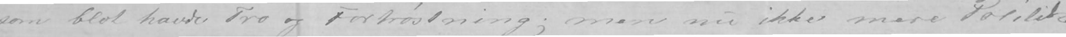som blot havde Tro og Fortrøstning; men nu ikke mere Politik
